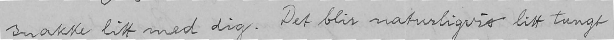snakke litt med dig. Det blir naturligvis litt tungt
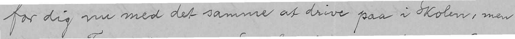for dig nu med det samme at drive paa i Skolen, men
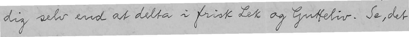dig selv end at delta i frisk Lek og Gutteliv. Se, det
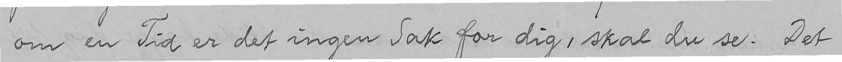om en Tid er det ingen Sak for dig, skal du se. Det
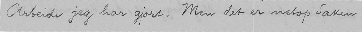Arbeide jeg har gjort. Men det er netop Saken
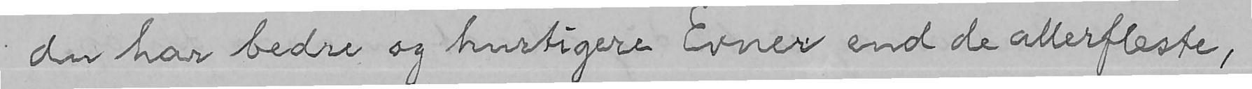du har bedre og hurtigere Evner end de allerfleste,
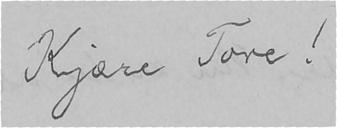Kjære Tore!
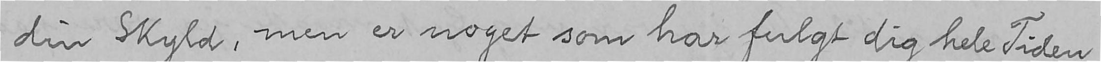din Skyld, men er noget som har fulgt dig hele Tiden
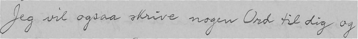Jeg vil ogsaa skrive nogen Ord til dig og
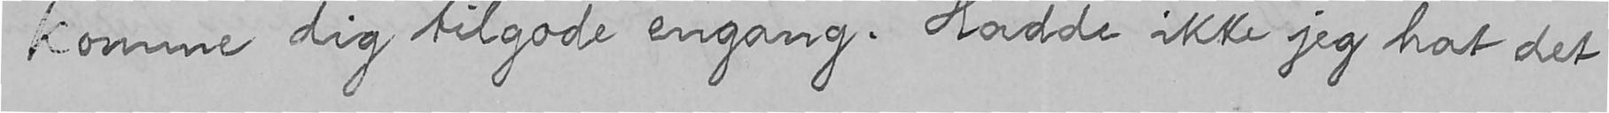komme dig tilgode engang. Hadde ikke jeg hat det
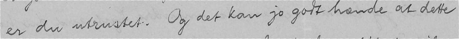er du utrustet. Og det kan jo godt hænde at dette
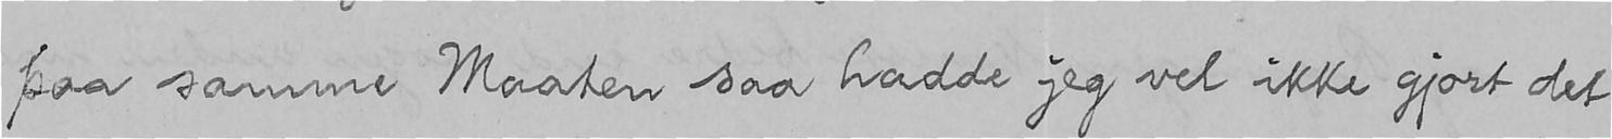paa samme Maaten saa hadde jeg vel ikke gjort det
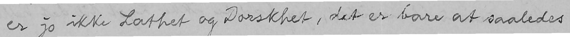er jo ikke Lathet og Dorskhet, det er bare at saaledes
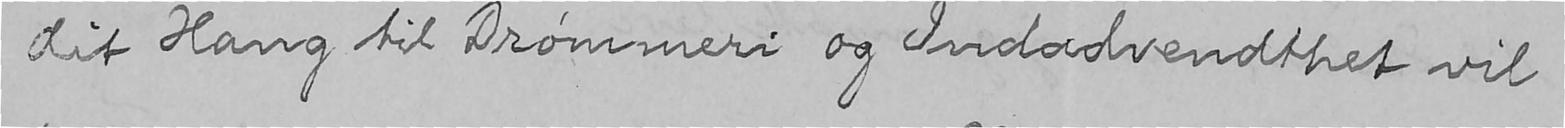dit Hang til Drømmeri og Indadvendthet vil
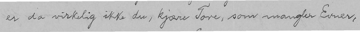er da virkelig ikke du, kjære Tore, som mangler Evner,
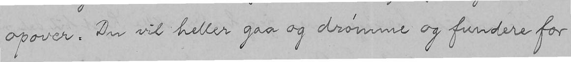opover. Du vil heller gaa og drømme og fundere for
